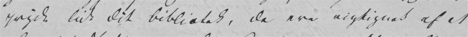pryde lidt Dit Bibliotek, de ere rigtignok af et
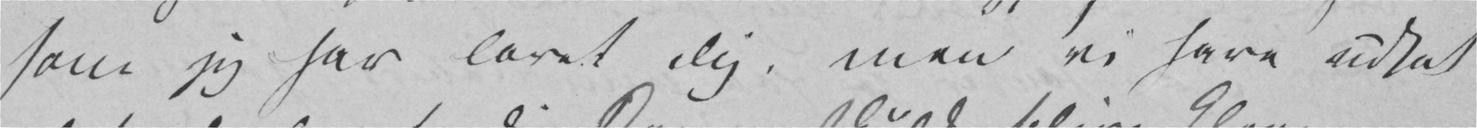som jeg har lovet Dig, men vi have udsat
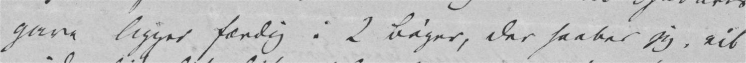ligger færdig i 2 Bøger, der haaber jeg, vil
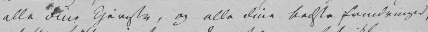alle Dine kjereste, og alle Dine bedste Erindringer,
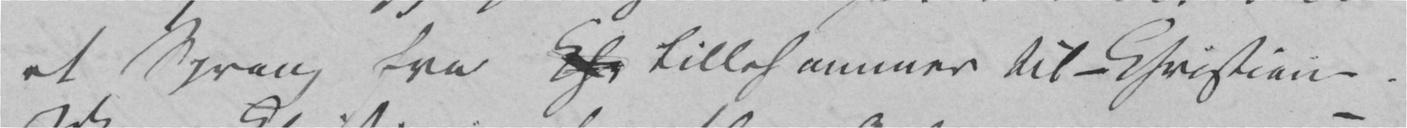et Sprang fra Chr Lillehammer til – Christiania.
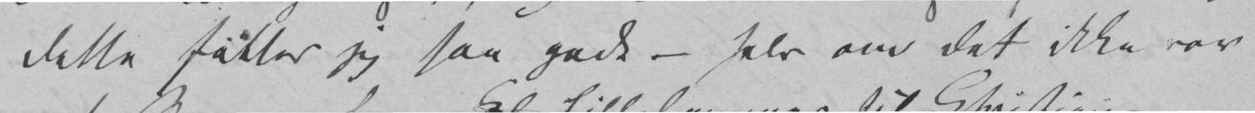dette fatter jeg saa godt – selv om det ikke var
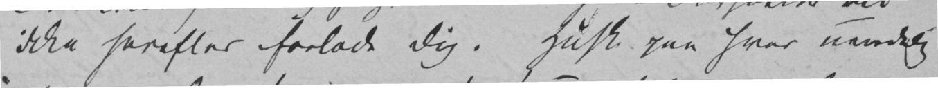ikke herefter forlade Dig. Husk paa hvor uendelig
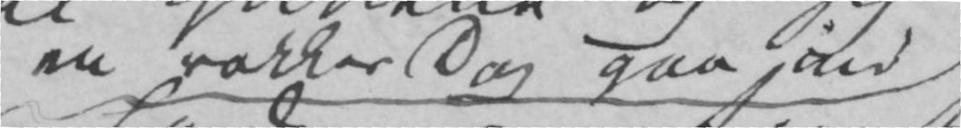en vakker Dag gaa ind
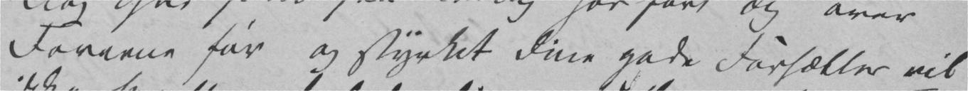Farerne før og styrket Dine gode Forsætter vil
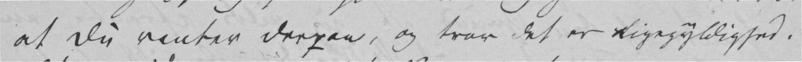at Du venter derpaa, og tror det er Ligegyldighed.
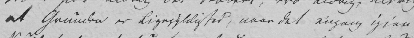at Grunden er Ligegyldighed, naar det engang igjen
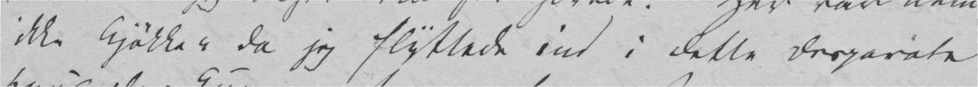ikke Kjøkken da jeg flyttede ind i dette desparate
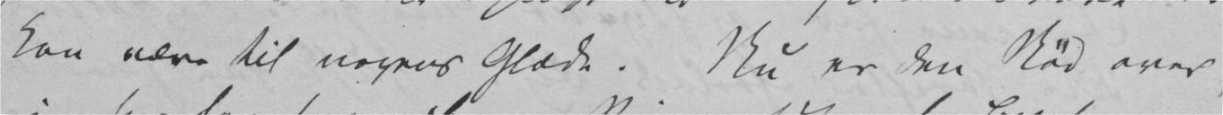kan være til nogens Glæde. Nu er den Nød over,
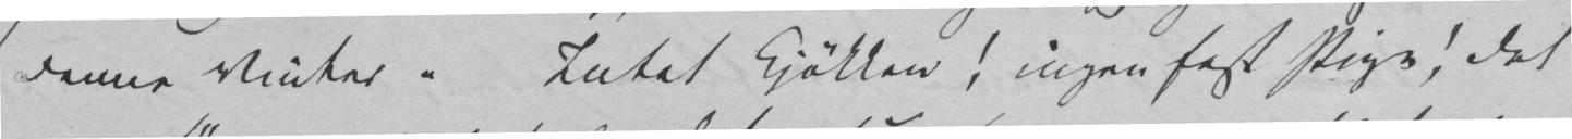denne Vinter. Intet Kjøkken! ingen fast Pige! det
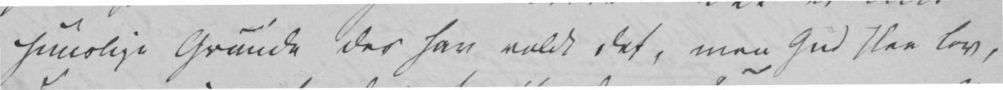huuslige Grunde der have voldt det, men Gud skee Lov,
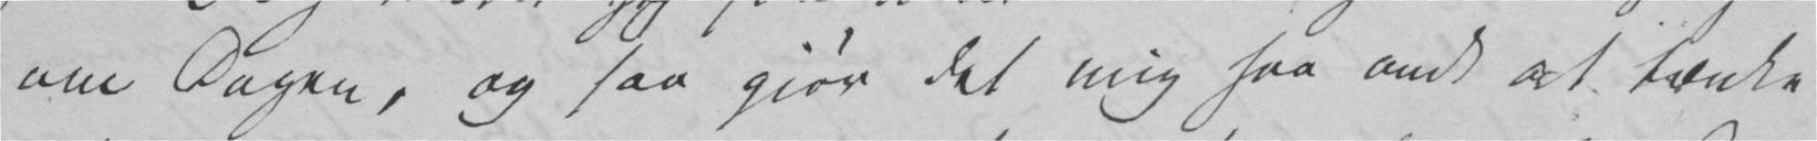om Dagen, og saa gjør det mig saa ondt at tænke
